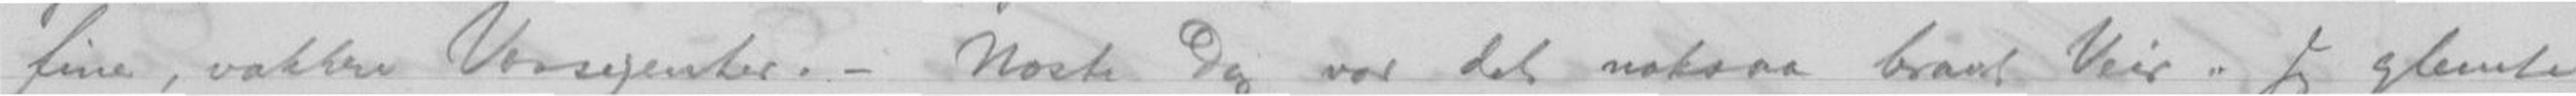fine, vakkre Vossejenter. - Næste Dag var det noksaa brad Veir. Jeg glemte
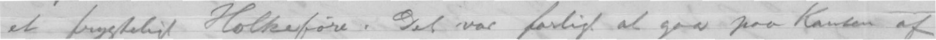et frykteligt Holkeføre. Det var farligt at gaa paa Kanten af
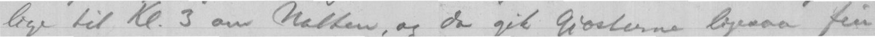lige til Kl. 3 om Natten, og da gik Gjæsterne ligesaa fin
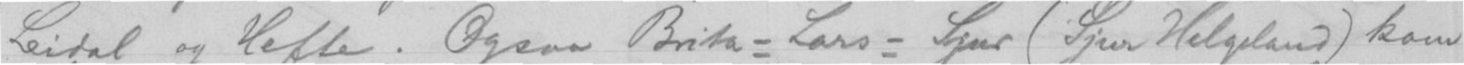Leidal og Hefte. Ogsaa Brita=Lars=Sjur (Sjur Helgeland) kom
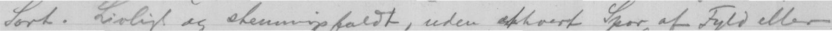Sort. Livligt og stemningsfuldt, uden ethvert Spor af Fyld eller
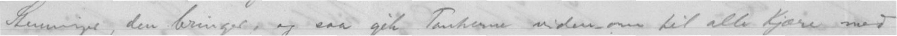Stemninger, den bringer, og saa gik Tankerne viden om til alle Kjære med
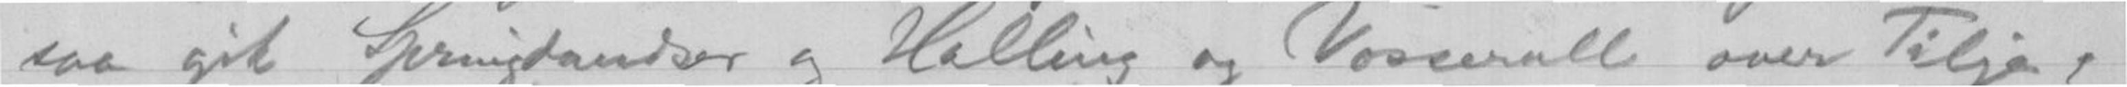saa gik Springdandser og Halling og Vosserull over Tilje, -
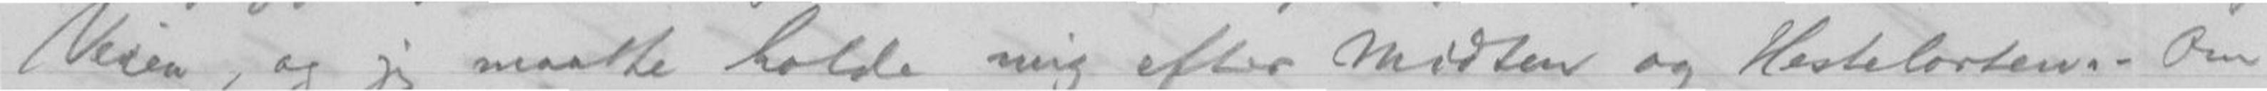Veien, og jeg maatte holde mig efter Midten og Hestelorten. Om
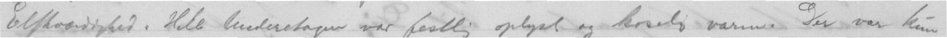Elskværdighed. Hele Underetagen var festlig oplyst og koselig varm. Der var kun
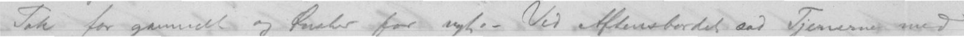Tak for gammelt og Ønsker for nyt. - Ved Aftensbordet sad Tjenerne med
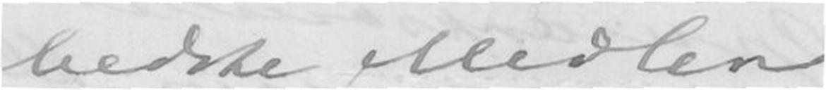bedste Midler
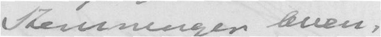Stemninger leven-
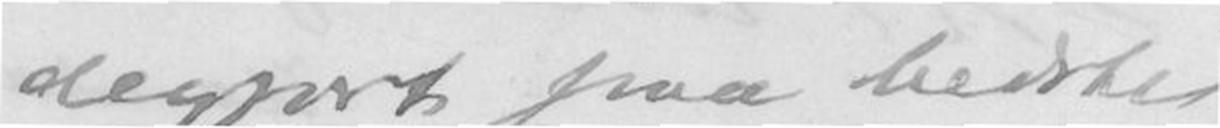degjort paa bedste
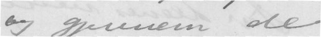og gjennem de
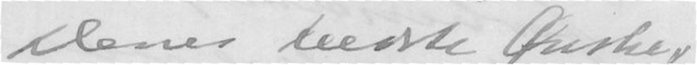Deres bedste Ønsker
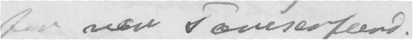fra vor Tonesadfærd.
